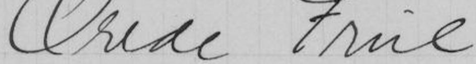Ærede Frue.
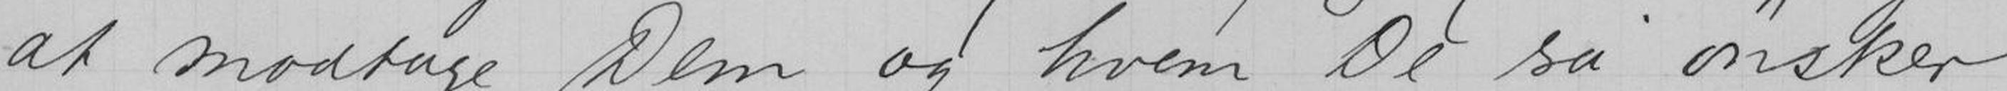at modtage Dem og hvem De så ønsker
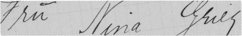Fru Nina Grieg
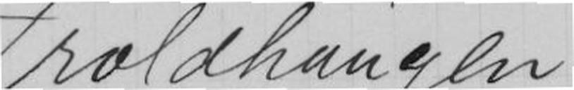Troldhaugen
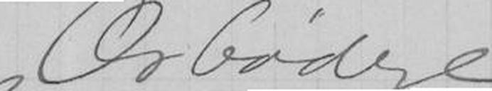Ærbødige
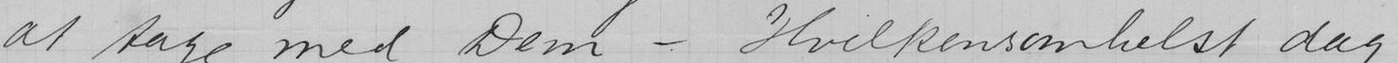at tage med Dem - Hvilkensomhelst dag
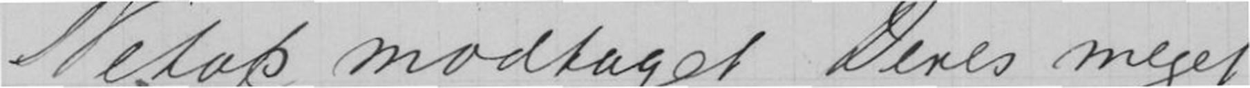Netop modtaget Deres meget
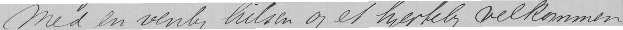Med en venlig hilsen og et hjertelig velkommen
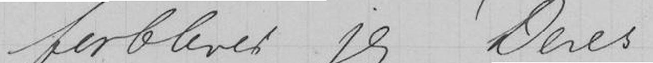forbliver jeg Deres
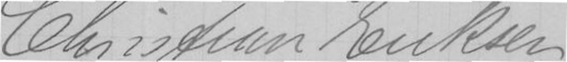Christian Eriksen
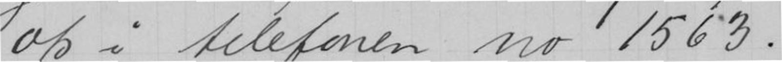op i telefonen no 1563.
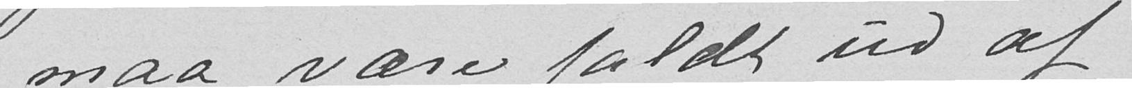maa være faldt ud af
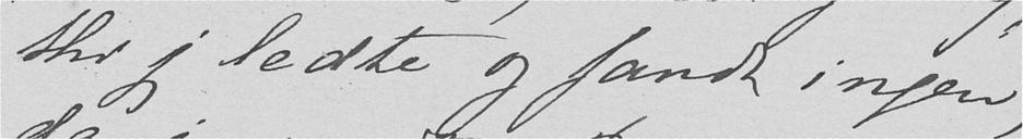thi jeg ledte og fandt ingen
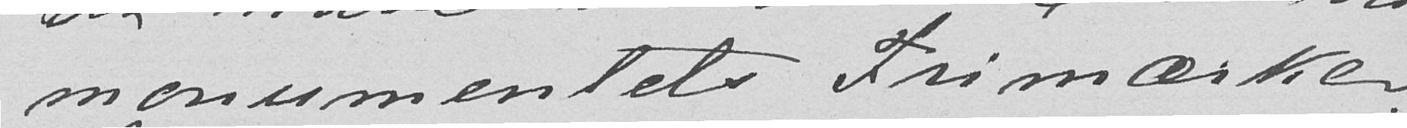monumentets Frimærker.
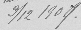3/12 1907.
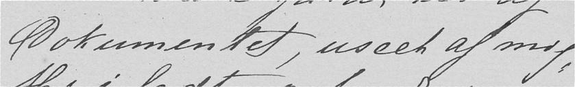Dokumentet, useet af mig,
